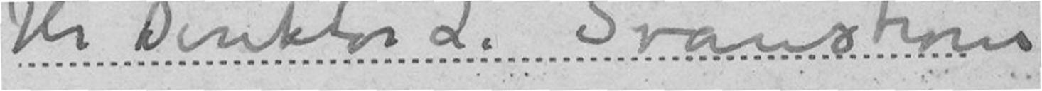Hr Direktør L. Svanström
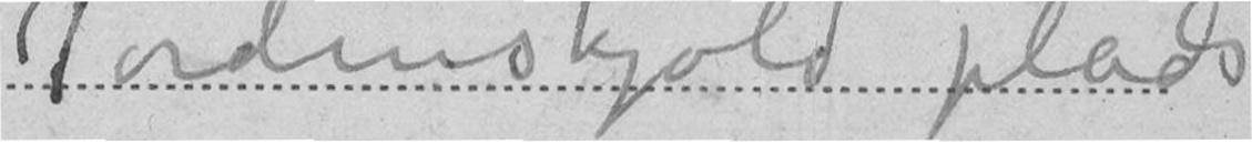Tordenskjold plads
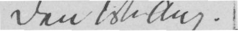den 1ste August
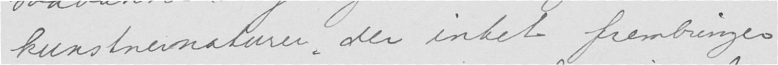kunstnernaturer, der intet frembringer
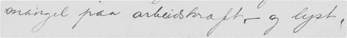mangel paa arbeidskraft, - og lyst,
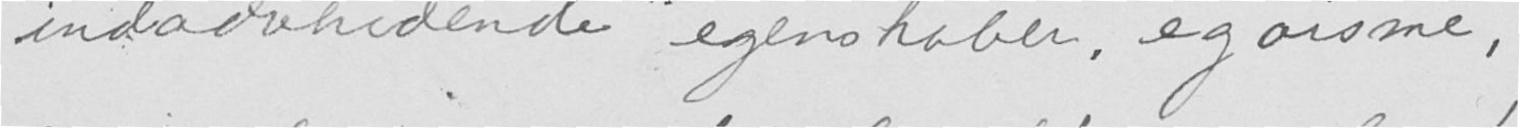"indadvenedende" egenskaber, egoisme,
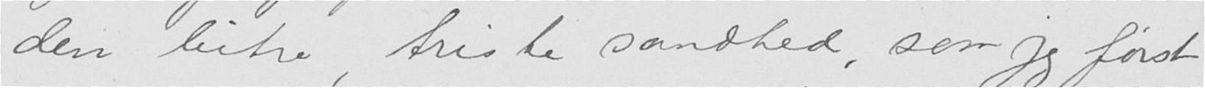den bitre, triste sandhed, som jeg først
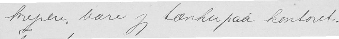krepere, bare jeg tænker paa kontoret.
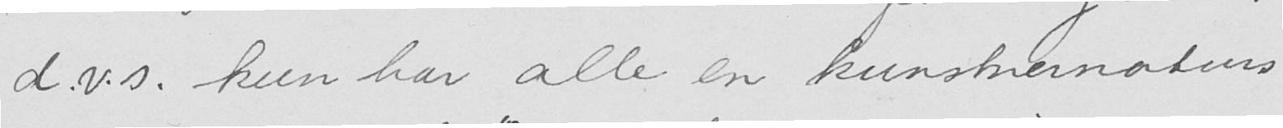d.v.s. kun har alle en kunstnernaturs
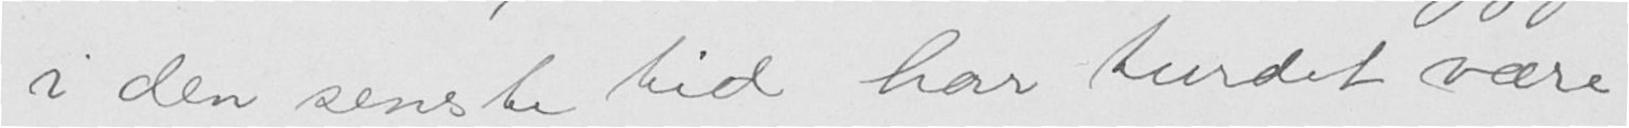i den seneste tid har turdet være
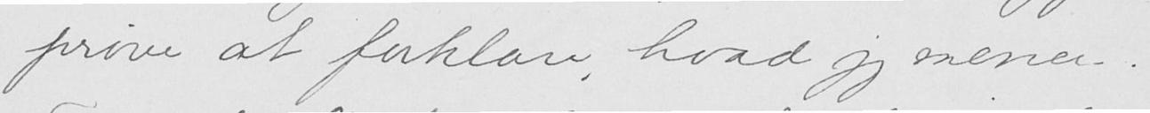prøve at forklare hvad jeg mener.
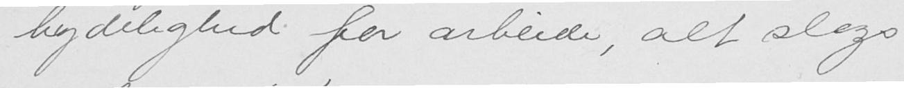bydelighed for arbeide, alt slags
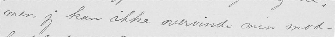men jeg kan ikke overvinde min mod-
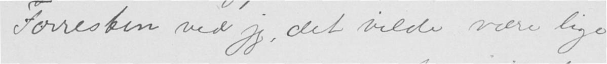Forresten ved jeg, det vilde være lige
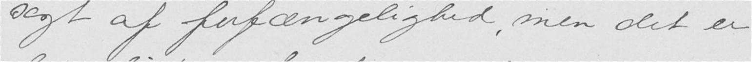sagt af forfængelighed, men det er
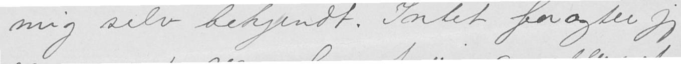mig selv bekjendt. Intet foragter jeg
